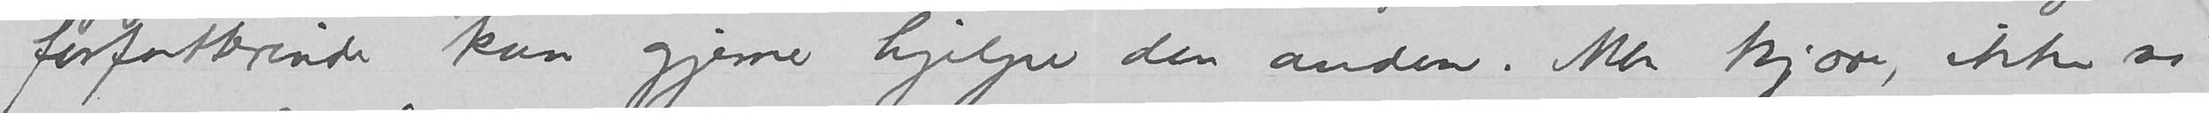forfatterinde kan gjerne hjelpe den anden. Men kjære, ikke si
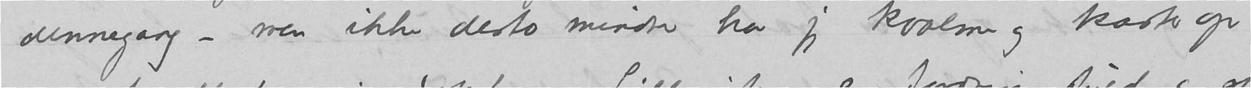dennegang – men ikke desto mindre har jeg kvalme og kaster op
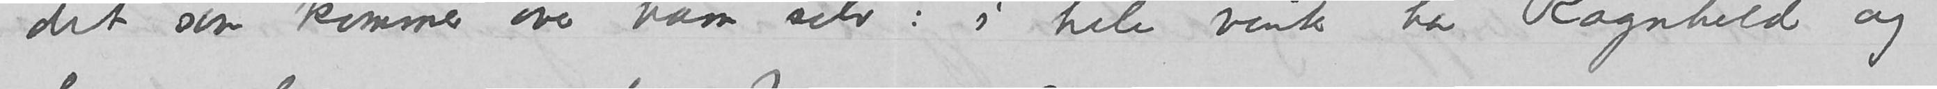det saa kommer over ham selv: i hele vinter har Ragnhild og
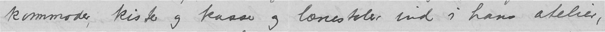kommoder, kister og kasser og lænestoler ind i hans atelier,
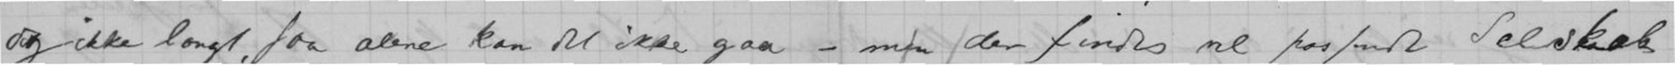dog ikke langt, saa alene kan det ikke gaa - men der findes vel passende Selskab
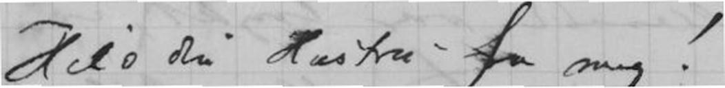Hils din Hustru fra mig!
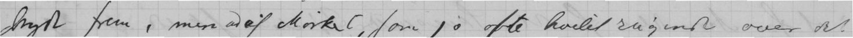bryde frem, mere ud af Mørket, som jo ofte hvilet rugende over det
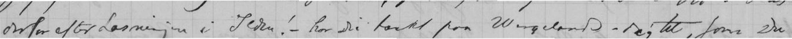derfor efter Lesningen i Ilden! - har Du tænkt paa Wergelands-digtet, som Du
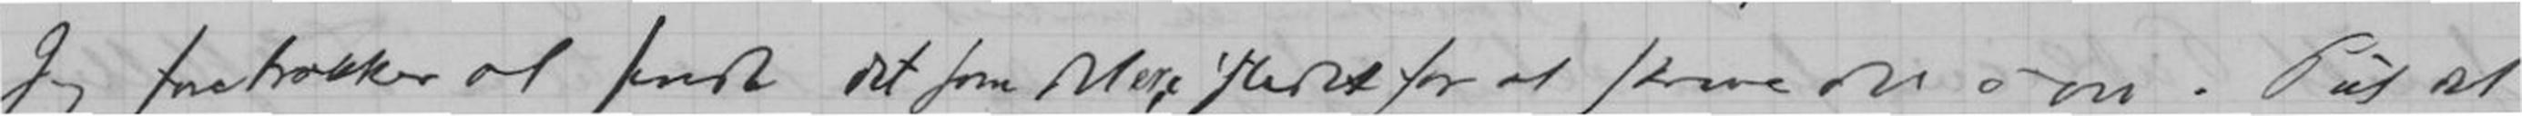Jeg foretrækker at sende det som det er, Istedet for at skrive det om. Put det
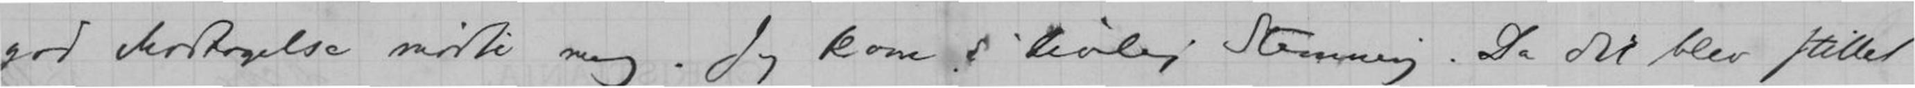god Modtagelse mødte mig. Jeg kom i livlig stemning. Da det blev stillet
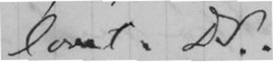lovet. DS.
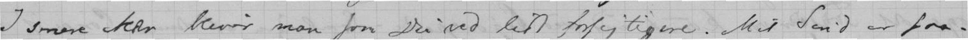I senere Alder bliver man som Du ved lidt forsigtigere. Mit Sind er saa-
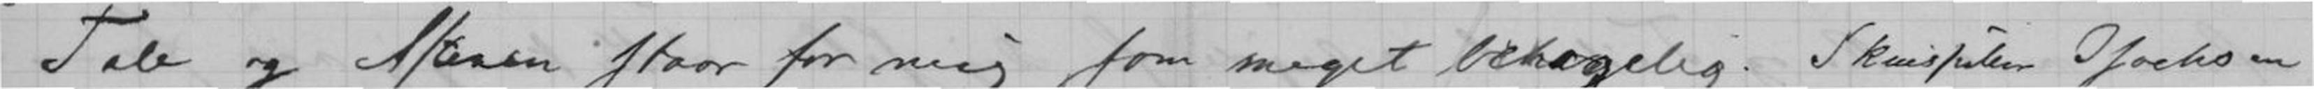Tale og Aftenen staar for mig som meget behagelig. Skuespiller Isachsen
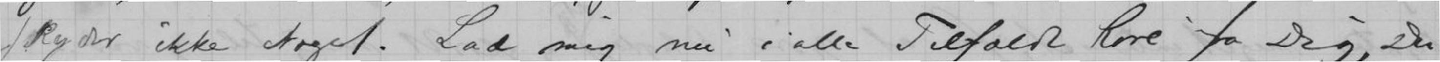skylder ikke Noget. Lad mig nu i alle Tilfælde høre fra Dig, Du
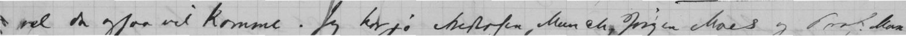ved du ogsaa vil komme. Jeg har jo Andersen, Munch, Jørgen Moes og Prof. Kon-
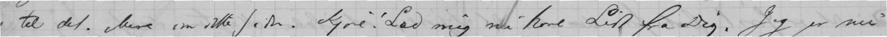til det. Mere om dette siden. Kjære! Lad mig nu høre Lidt fra Dig. Jeg er nu
